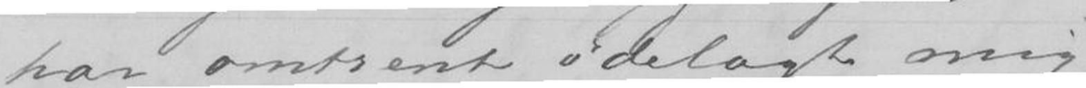har omtrent ødelagt mig
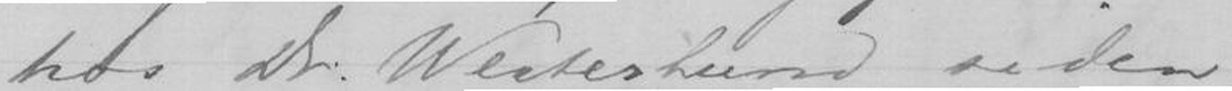hos Dr.Westerlund siden
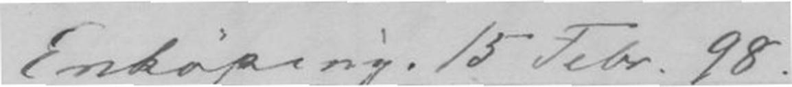Enköping. 15 Febr. 98.
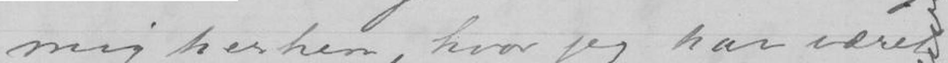mig herhen, hvor jeg har været
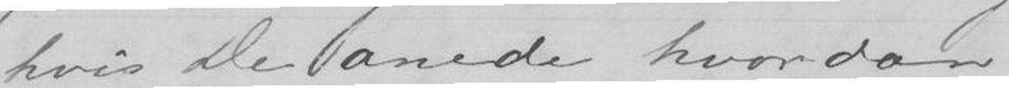hvis De anede hvordan
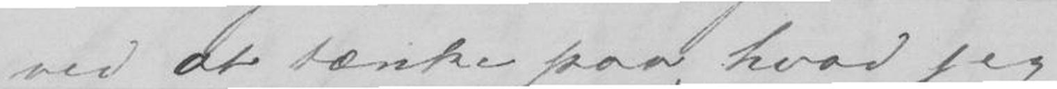ved at tænke paa, hvad jeg
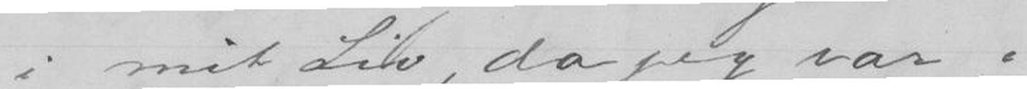i mit Liv, da jeg var i
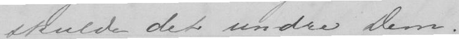skulde det undre Dem.
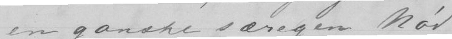en ganske særegen Nød
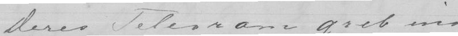Deres Telegram greb ind
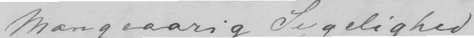Mangeaarig Sygelighed
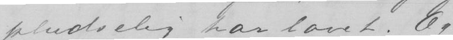pludselig har lovet. Og
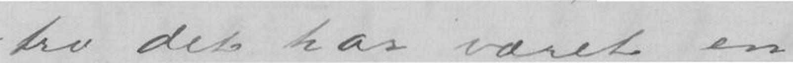tro det har været en
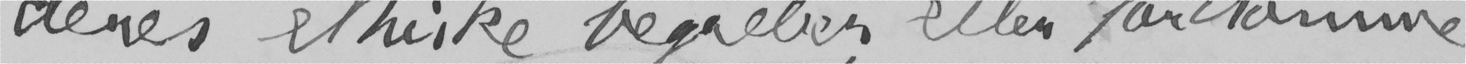deres ethiske begreber eller fordomme
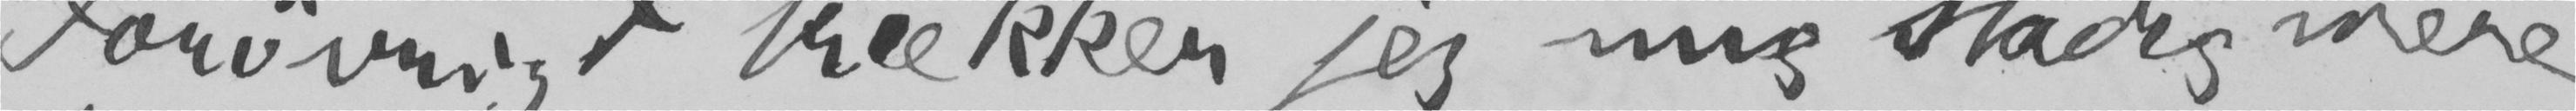Forøvrigt trækker jeg mig stadig mere
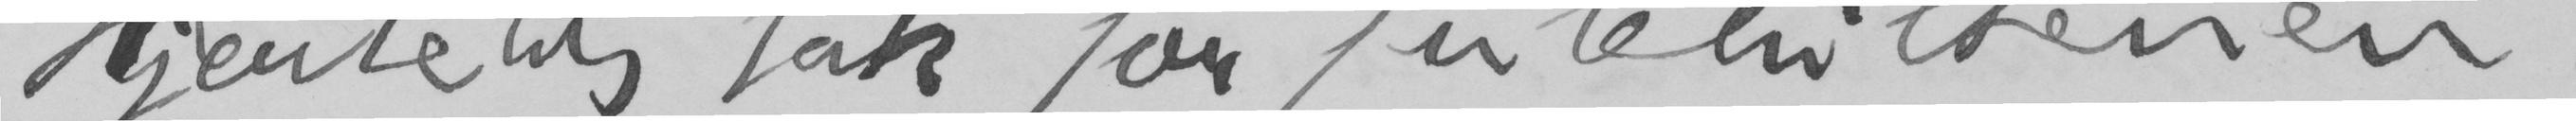Hjertelig tak for julehilsenen!
